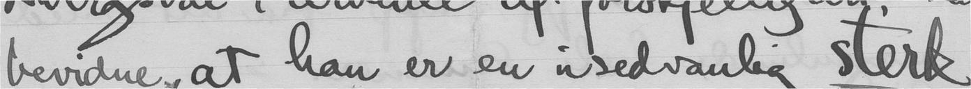bevidne, at han er en usedvanlig sterk
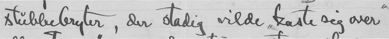stubbebryter, der stadig vilde "kaste sig over"
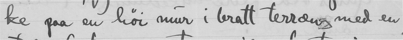ke paa en høi mur i bratt terræng med en
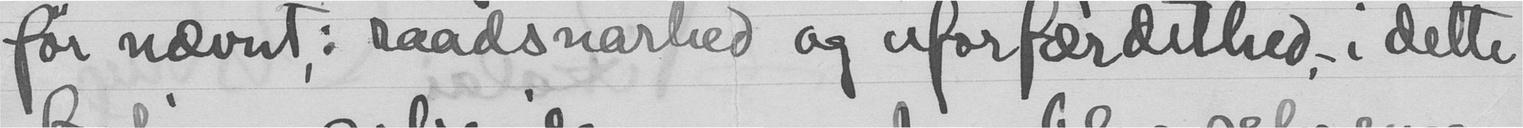før nævnt: raadsnarhed og uforfærdethed, - i dette
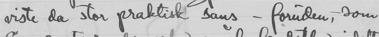viste da stor praktisk sans - foruden, - som
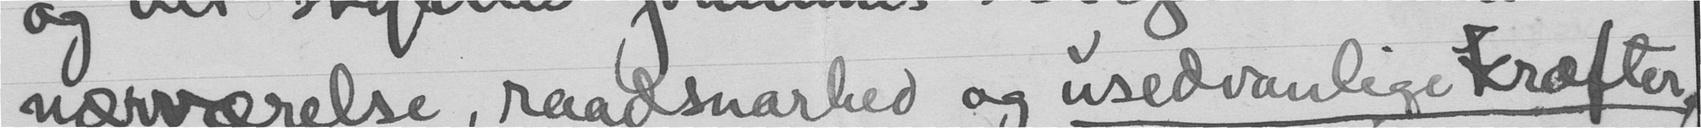nærværelse, raadsnarhed og usedvanlige kræfter.
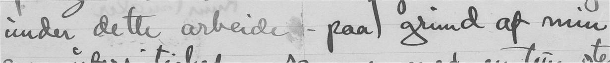under dette arbeide - paa grund af min
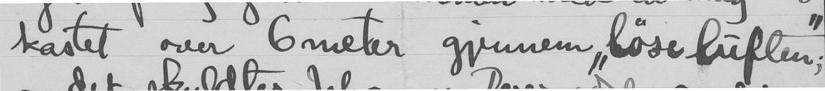kastet over 6 meter gjennem "løse luften";
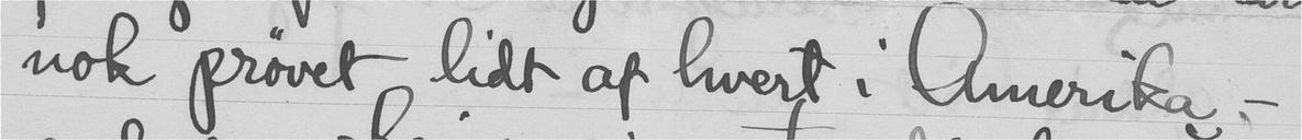nok prøvet lidt af hvert i Amerika, -
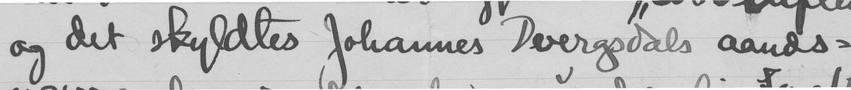og det skyldtes Johannes Dvergsdals aands-
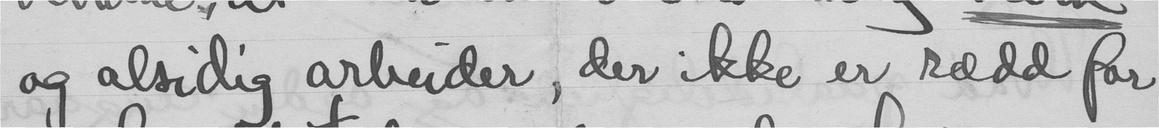og alsidig arbeider, der ikke er rædd for
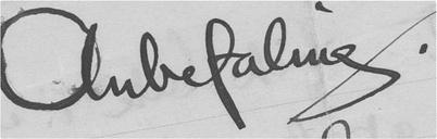Anbefaling.
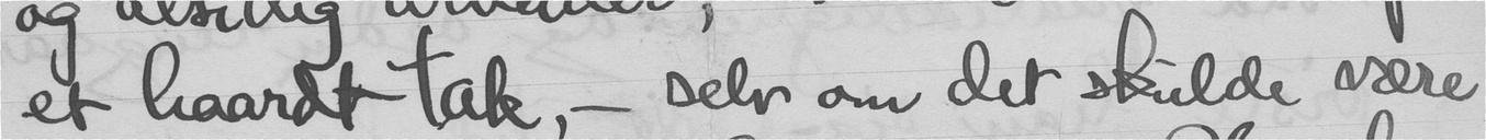et haardt tak, - selv om det skulde være
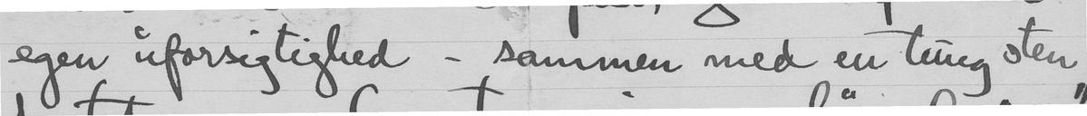egen uforsigtighed - sammen med en tung sten
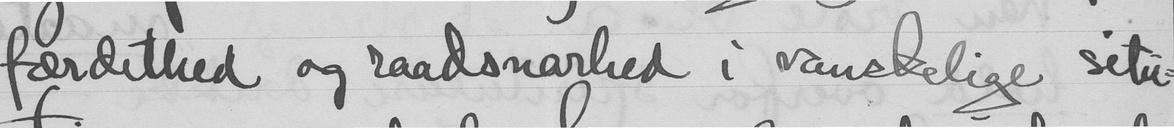færdethed og raadsnarhed i vanskelige situ-
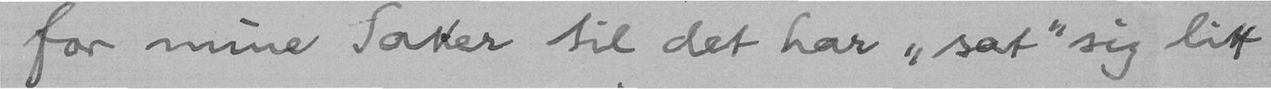for mine Saker til det har "sat" sig litt
